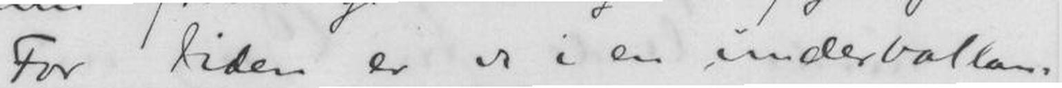For tiden er vi i en underballan-
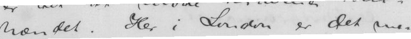hændet. Her i London er det me-
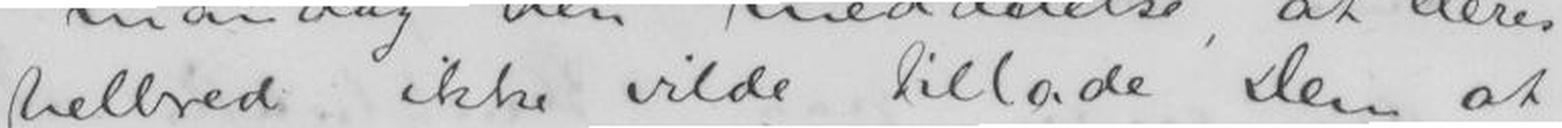helbred ikke vilde tillade Dem at
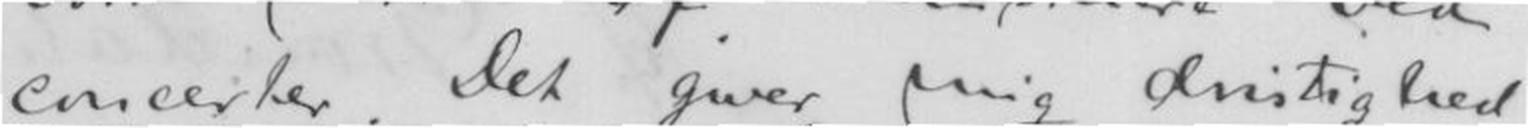concerter. Det giver mig dristighed
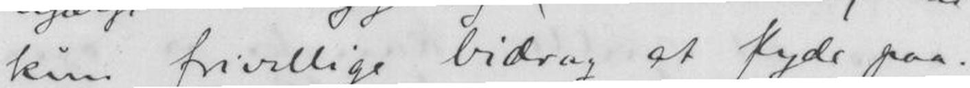kun frivillig bidrag at flyde paa.
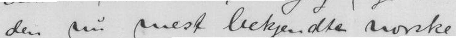den nu mest bekjendte norske
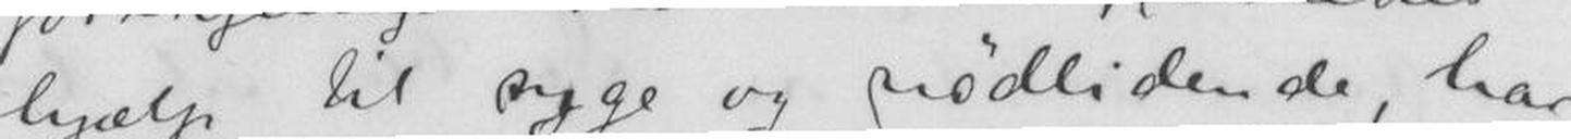hjælp til syge og nødlidende, har
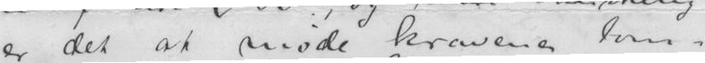er det at møde kravene tom-
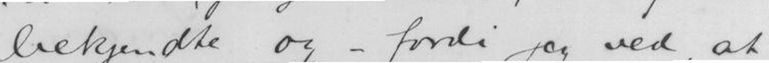bekjendte og - fordi jeg ved, at
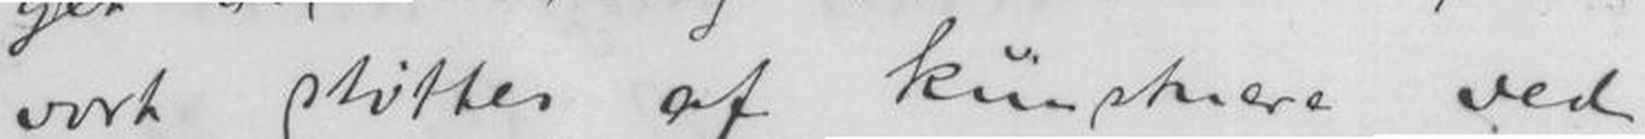vort støtter af kunstnere ved
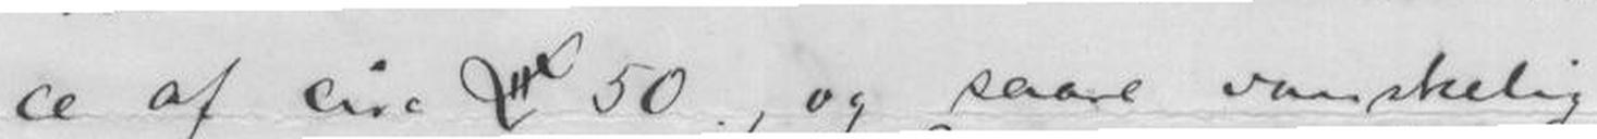ce af circ. £ 50, og saare vanskelig
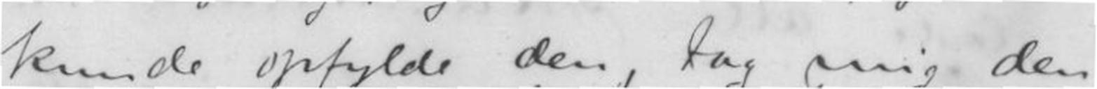kunde opfylde den, tag mig den
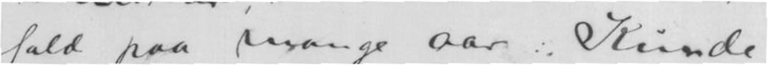fald paa mange aar. Kunde
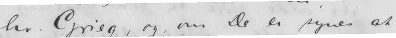hr. Grieg, og om De ei synes at
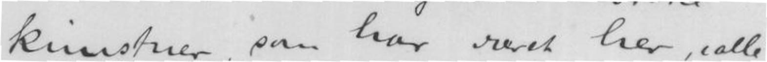kunstner, som har været her, ialle
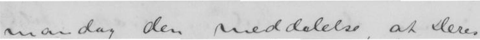mandag den meddelelse, at Deres
